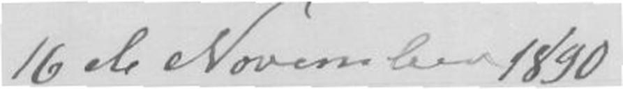16 de November 1890
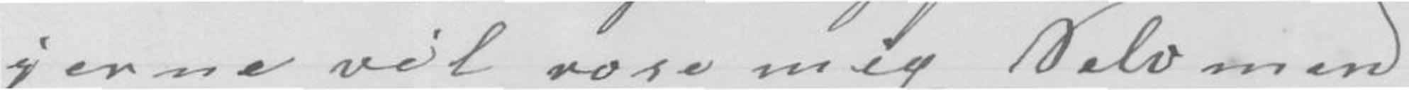jerne vil rose mig Selv men
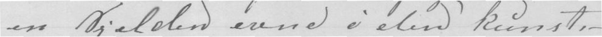en Sjelden evne i den kunst, -
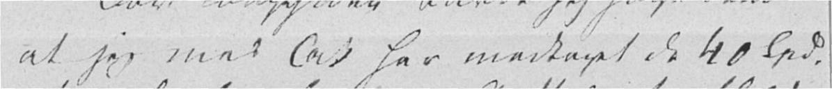at jeg med Tak har modtaget de 40 Spd.
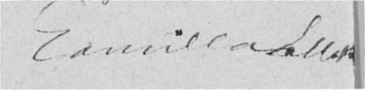Camilla Collett
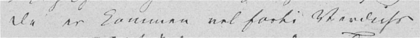De er kommen vel forbi Verdichs
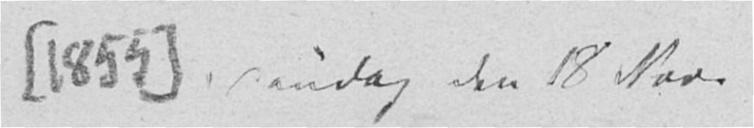Søndag den 18 Nov.
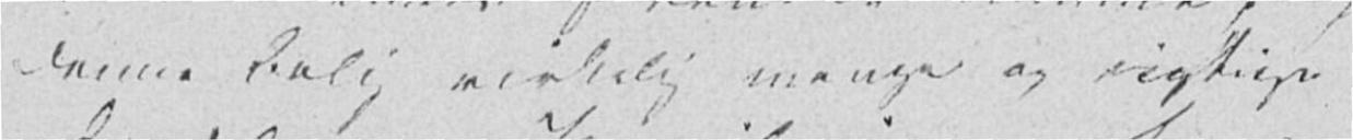denne Bolig virkelig mange og rigtige
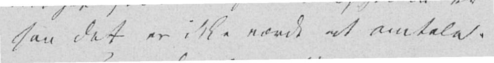saa det er ikke værdt at omtale.
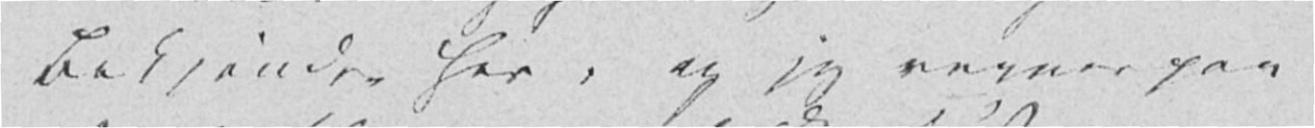Bekjendte her, og jeg regner paa
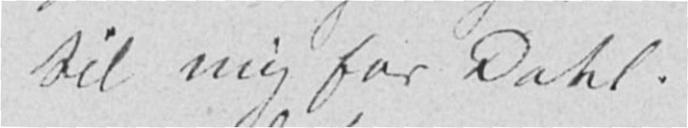til mig for Dahl.
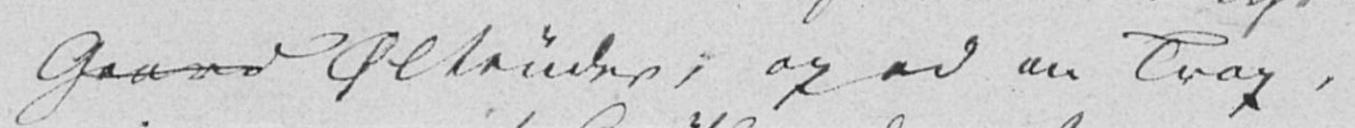Gaard Øltønder; op ad en Trap,
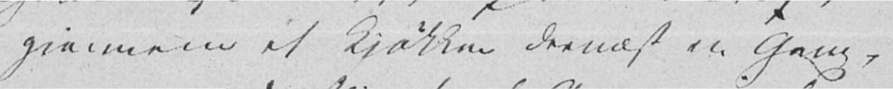gjennem et Kjøkken dernæst en Gang,
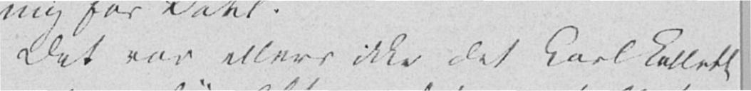Det var ellers ikke det Carl Collett
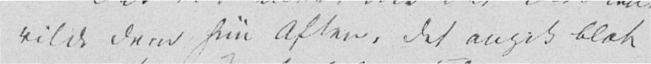vilde Dem hiin Aften, det angik blot
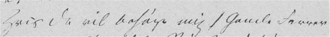Hvis De vil besøge mig (Gamle Farver
